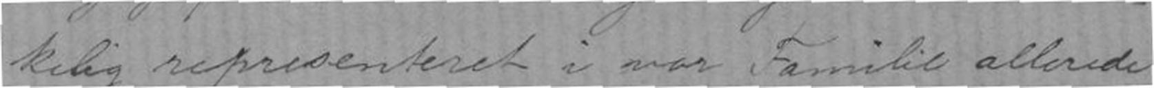kelig representeret i vor Familie allerede
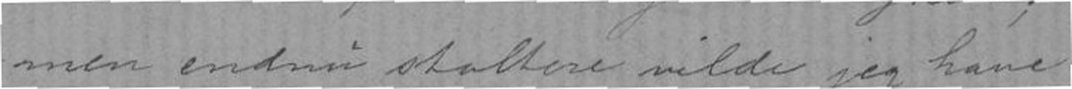men endnu stoltere vilde jeg have
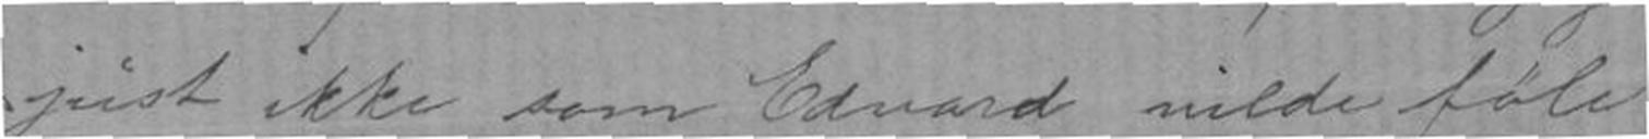just ikke som Edvard vilde føle
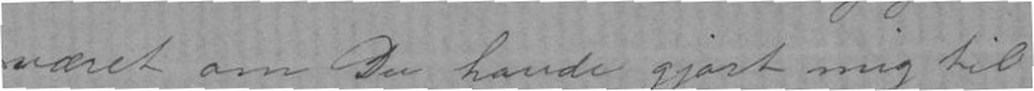været om Du havde gjort mig til
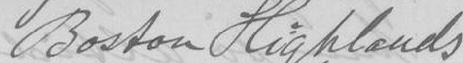Boston Highland
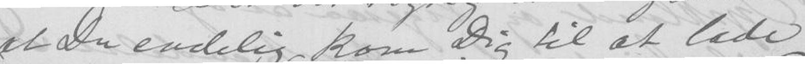at Du endelig kom Dig til at lade
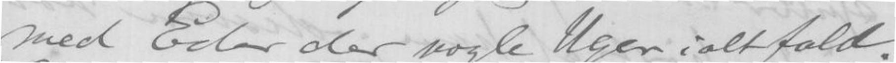med Eder der nogle Uger ialtfald.
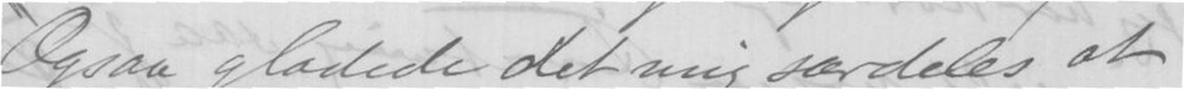Ogsaa glædede det mig særdeles at
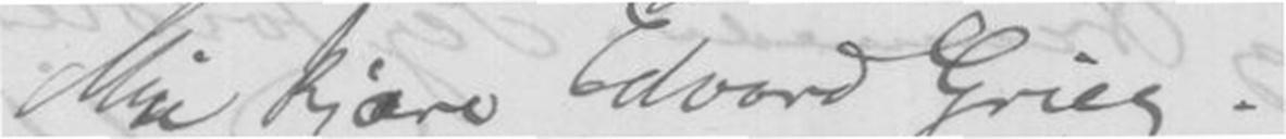Min kjære Edvard Grieg.
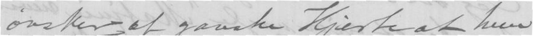og ønsker af ganske Hjerte at hun
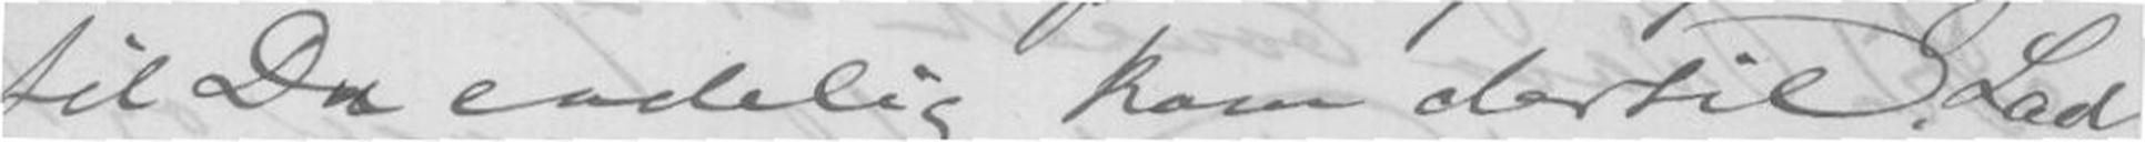til Du endelig kom dertil. Lad
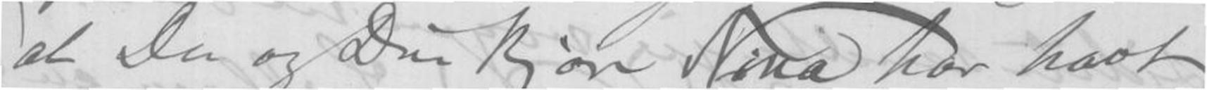at Du og Din kjære Nina har havt
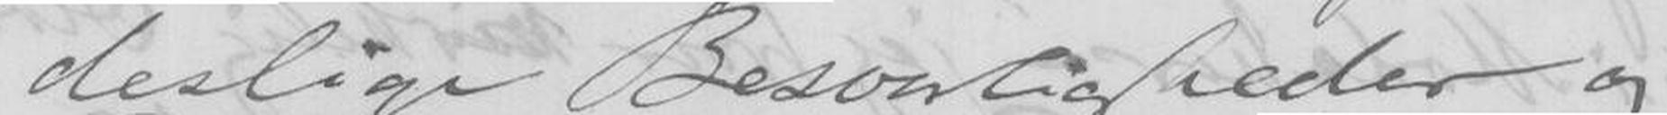deslige Besverligheder og
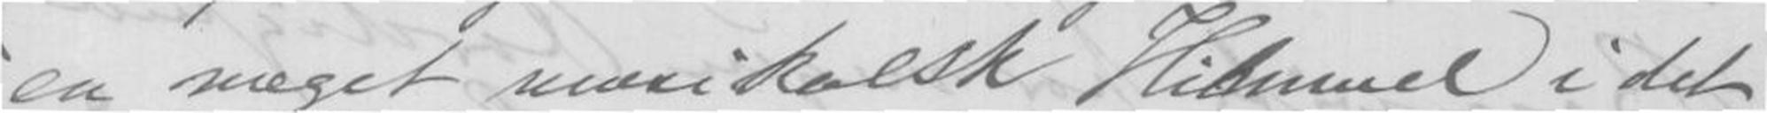en meget musikalsk Himmel i det
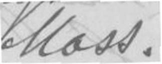Mass.
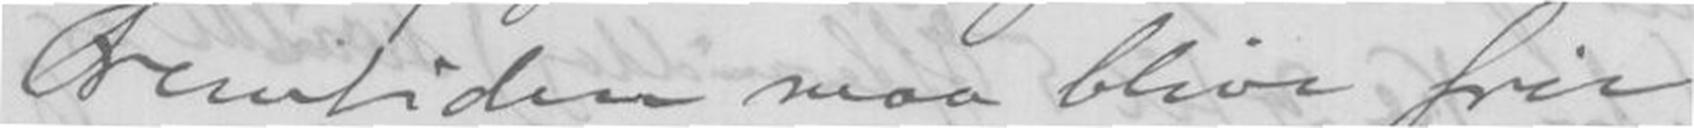Fremtiden maa blive frie
